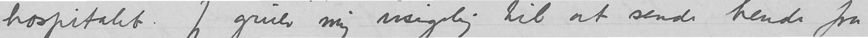hospitalet. Jeg gruer mig usigelig til at sende hende fra
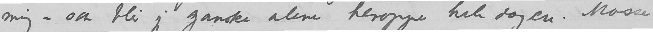mig – saa blir jeg ganske alene heroppe hele dagen. Mosse
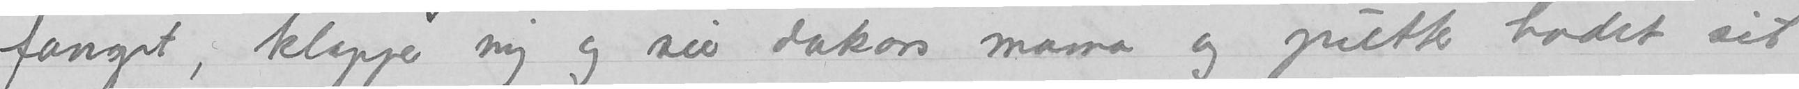fanget, klapper mig og sier dakars mama og putter hodet sit
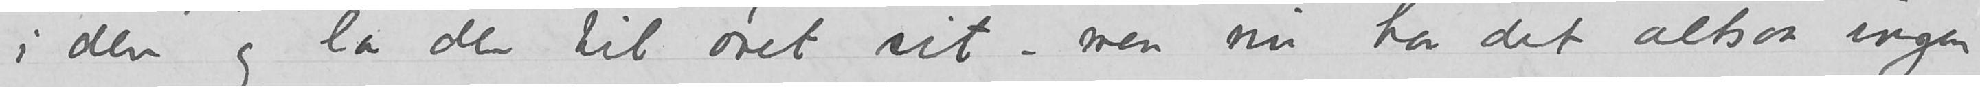i den og la den til øret sitt – men nu har det altsaa ingen-
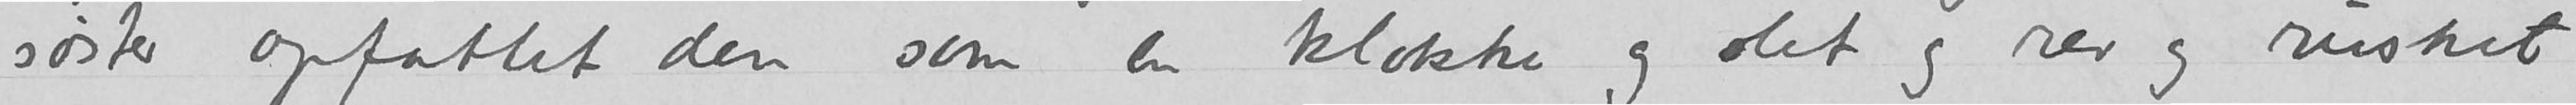søster opfattet den som en klokke og slet og rev og rusket
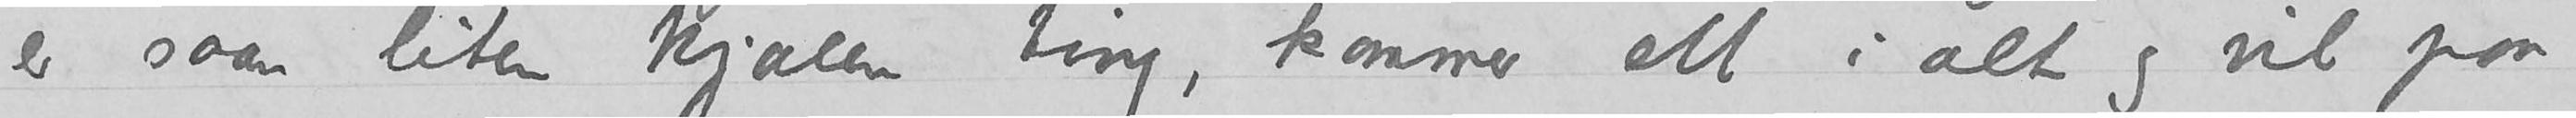er saan liten kjælen ting, kommer ett i alt og vil paa
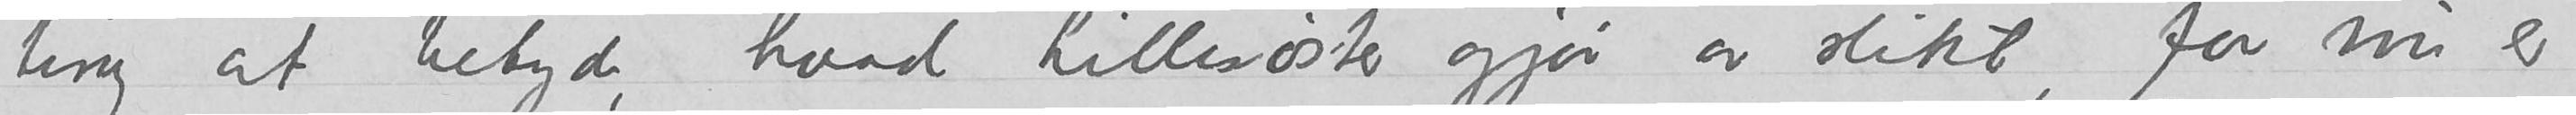ting at betyde, hvad Lillesøster gjør av slikt, for nu er
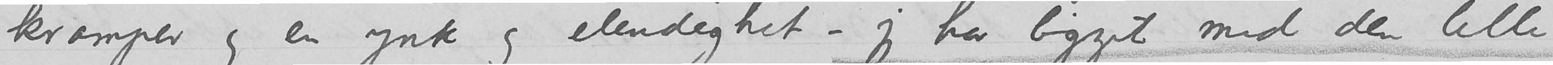kramper og en ynk og elendighet – jeg har ligget med den lille
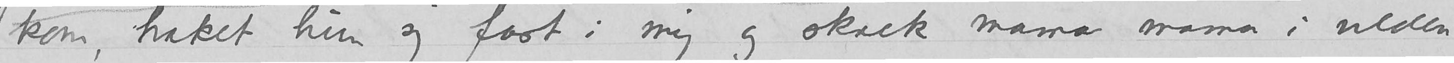kom, haket hun sig fast i mig og skrek mama mama i vilden
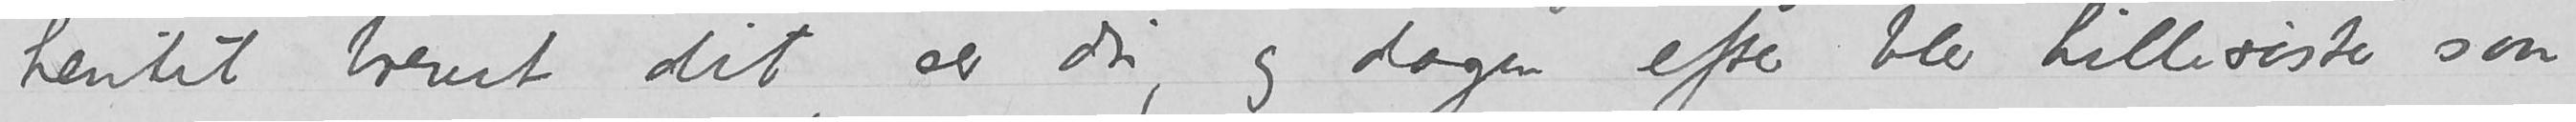hentet brevet dit ser du, og dagen efter blev Lillesøster saa
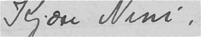Kjære Nini,
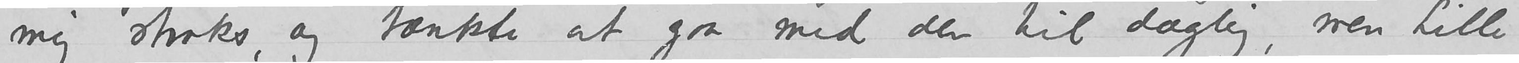mig straks, og tænkte at gaa med den til daglig, men Lille-
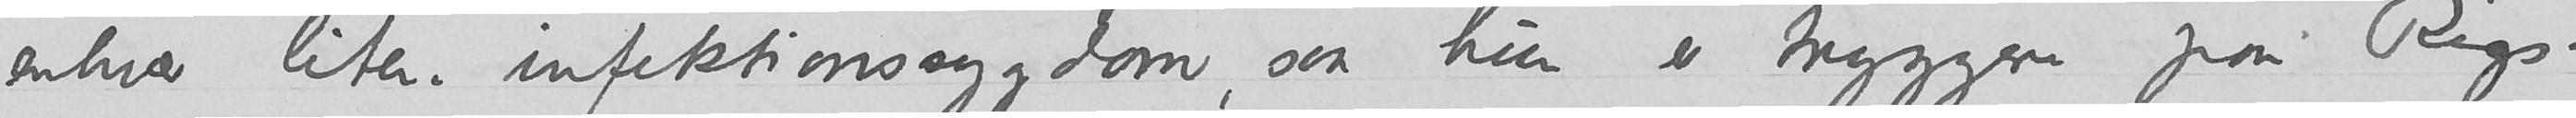enhvær liten infektionssygdom, saa hun er tryggere paa Rigs-
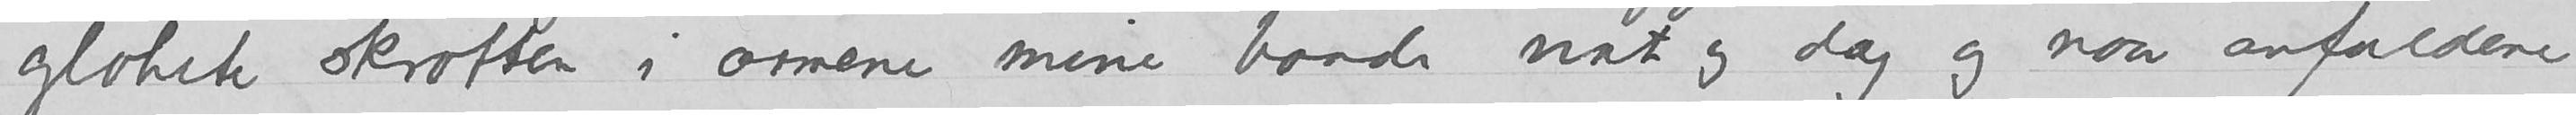glohete skrotten i armene mine baade nat og dag og naar anfaldene
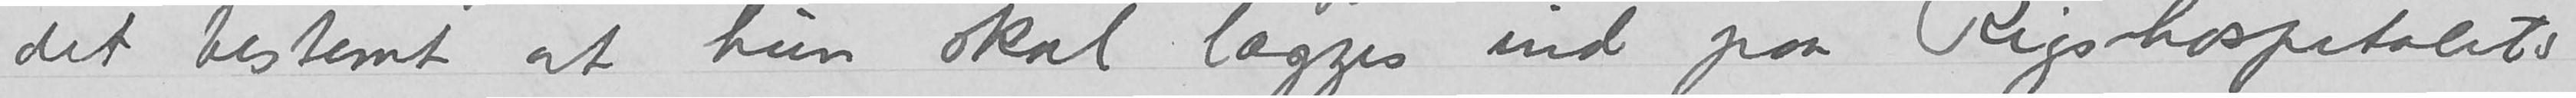det bestemt at hun skal lægges ind paa Rigshospitalets
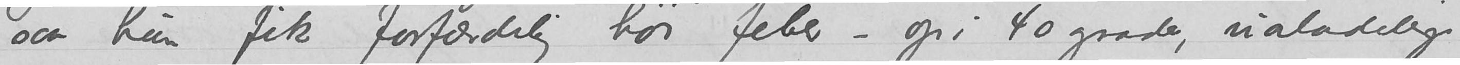saa hun fik forfærdelig høi feber – opi 40 grader, ualadelige
